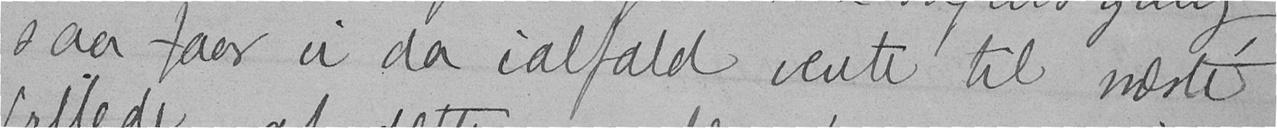saa faar vi da ialfald vente til næste
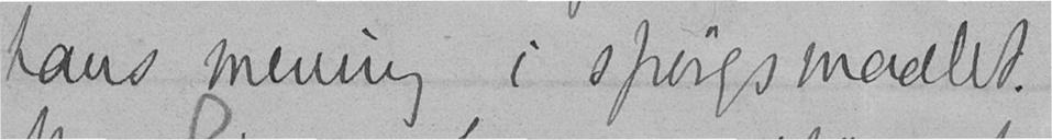hans mening i spørgsmaalet.
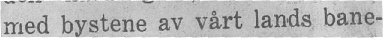med bystene av vårt lands bane-
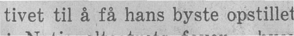tivet til å få hans byste opstillet
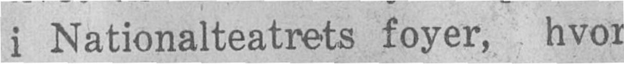i Nationalteatrets foyer, hvor
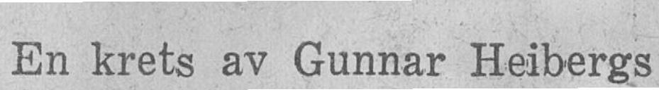En krets av Gunnar Heibergs
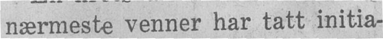nærmeste venner har tatt initia-
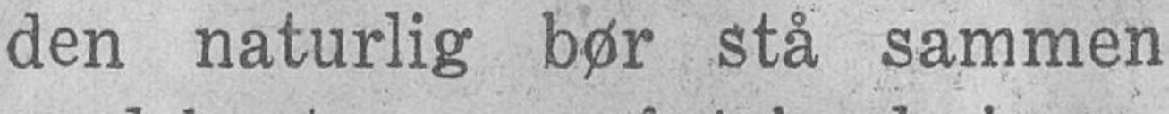den naturlig bør stå sammen
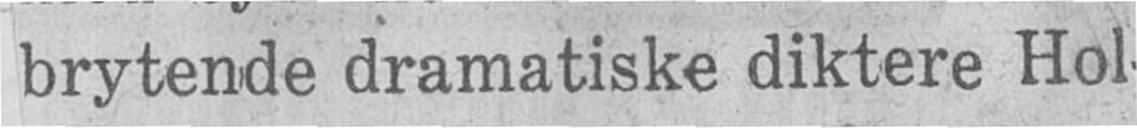brytende dramatiske diktere Hol-
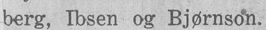berg, Ibsen og Bjørnson.
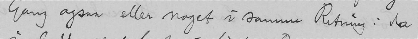Gang ogsaa eller noget i samme Retning: da
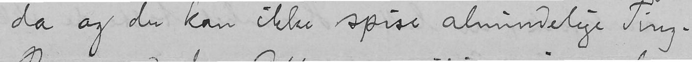da og du kan ikke spise almindelige Ting.
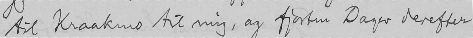til Kraaknes til mig, og fjorten Dager derefter
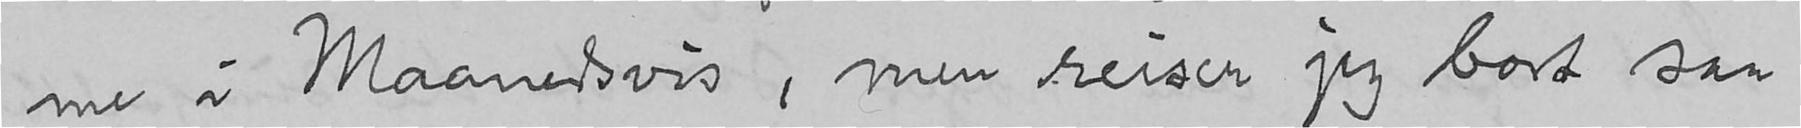me i Maanedsvis, men reiser jeg bort saa
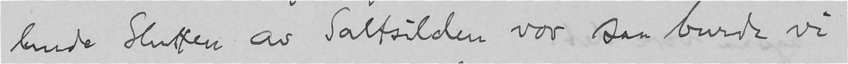lunde Slutten av Saltsilden vor saa burde vi
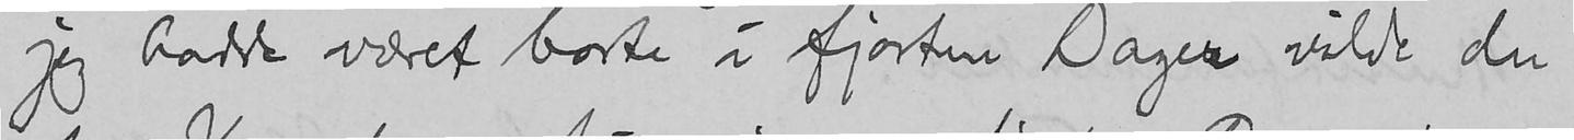jeg hadde været borte i fjorten Dager vilde du
